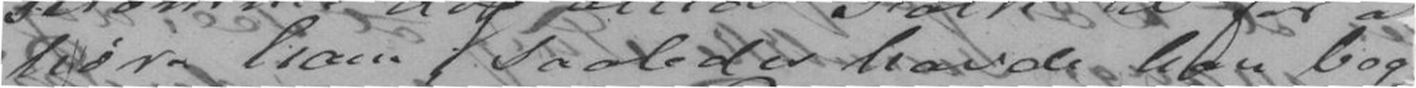høre ham. Saaledes havde han beg
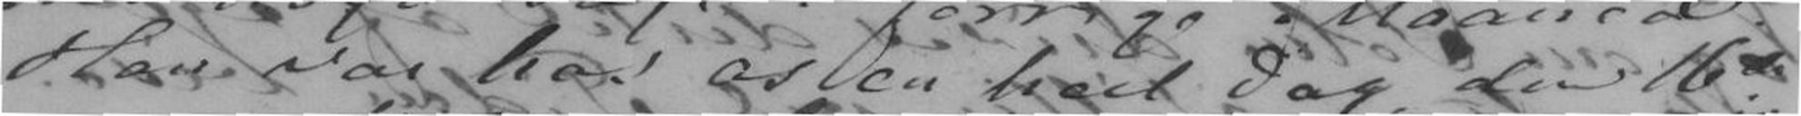Han var hos os en heel Dag den 16de
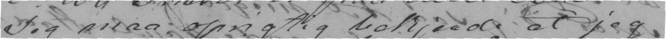Jeg maa oprigtig bekjende at jeg
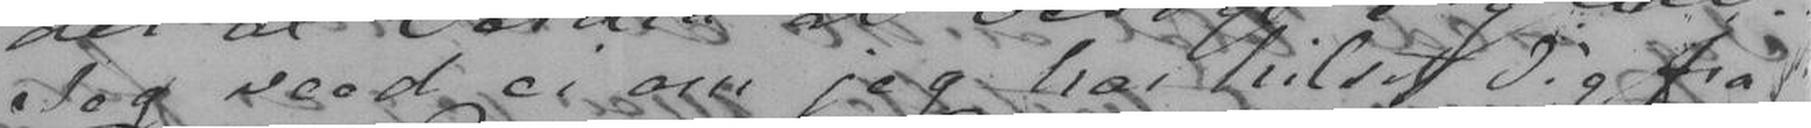Jeg veed ei om jeg har hilset Dig fra
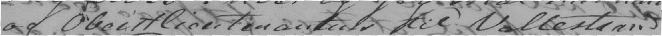og Oberstlieutenantens til Vallestrand
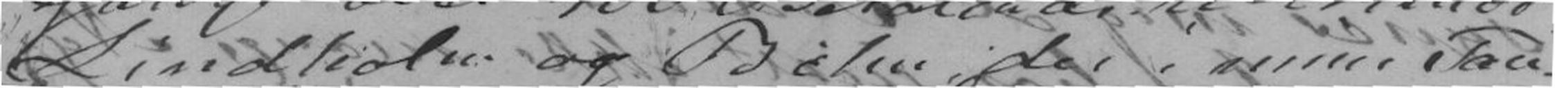Lindholm og Bøhn der i mine Tan-
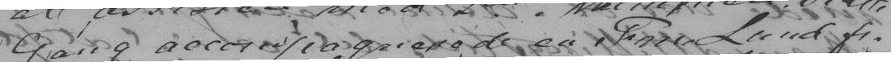Gang accompagnerede en Fru Lund fra
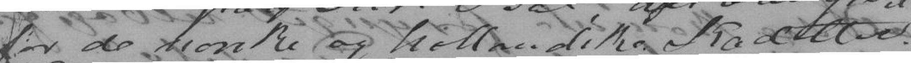for de norske og hollandske Kadetter
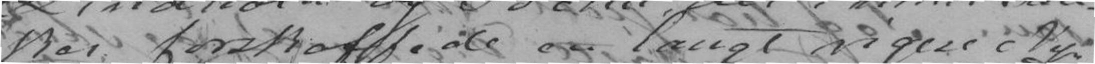ker forskaffede en langt rigere Ny-
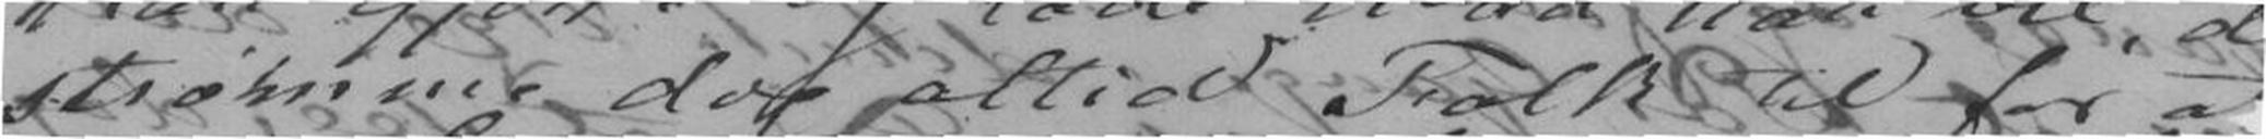strømme dog altid Folk til for a
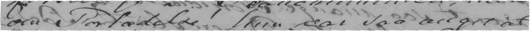om Forladelse! hun var saa angst at
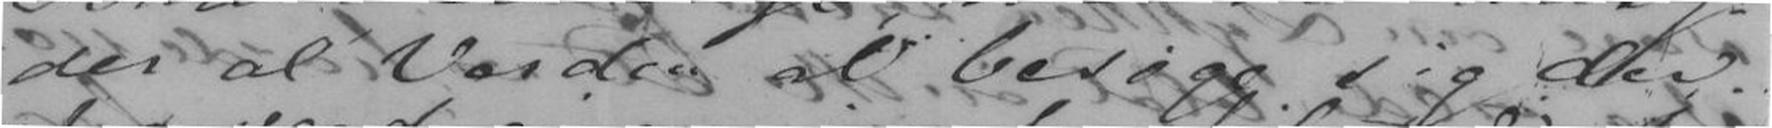der al Verden at besøge sig der.
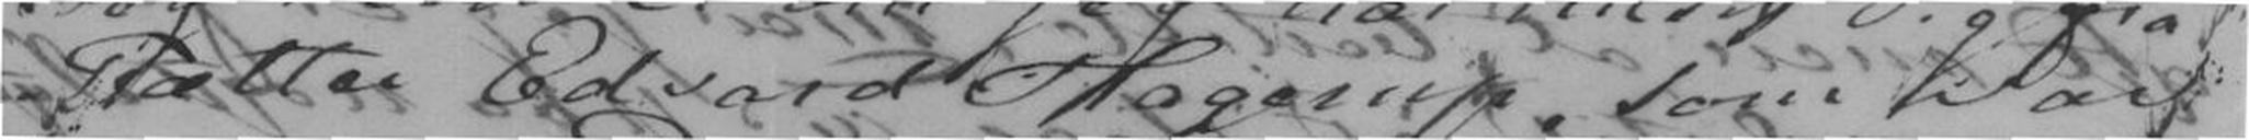Fætter Edvard Hagerup, som var
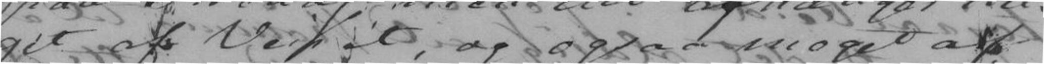get af Veiret, og ogsaa meget af
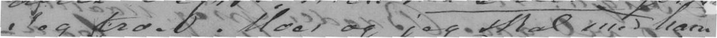Jeg troer Moer og jeg skal med ham
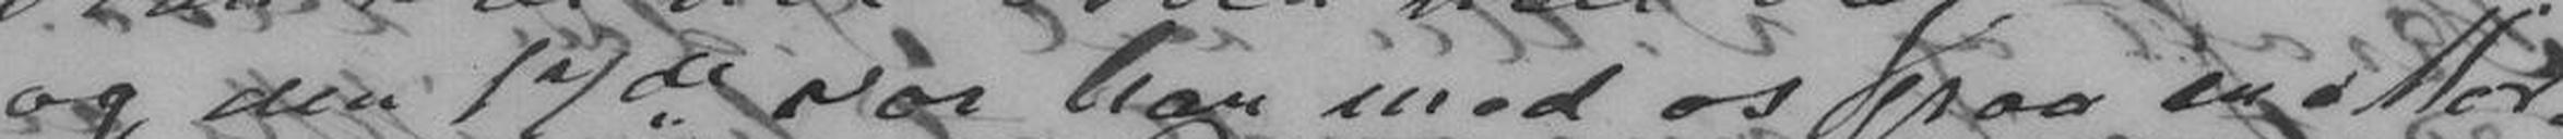og den 17de var han med os paa en Mor-
